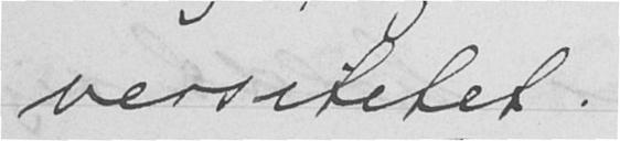versitetet.
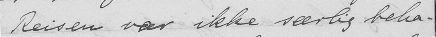Reisen var ikke særlig beha-
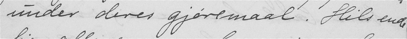under deres gjøremaal. Hils ende-
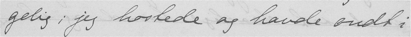gelig; jeg hostede og havde ondt i
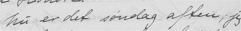Nu er det søndag aften; jeg
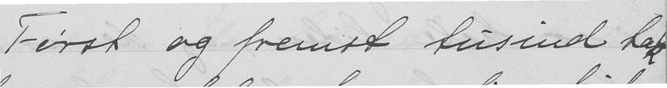Først og fremst tusind tak
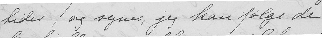tider, og synes, jeg kan følge de
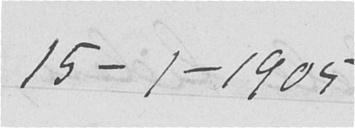15-1-1904
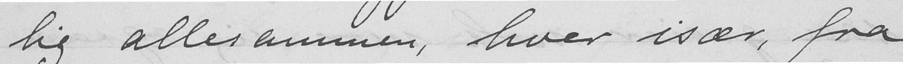lig allesammen, hver især, fra
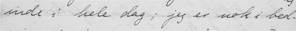inde i hele dag; jeg er nok i bed-
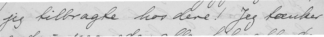jeg tilbragte hos dere! Jeg tænker
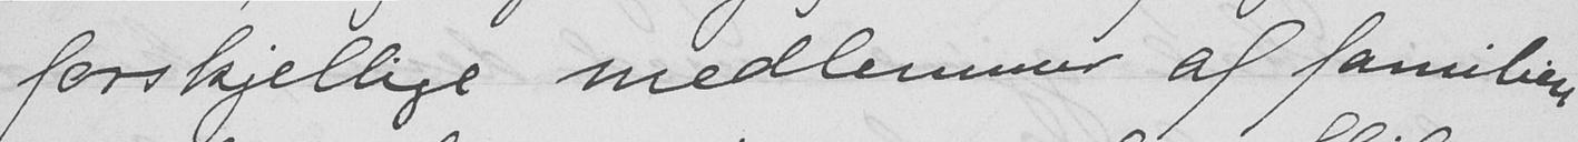forskjellige medlemmer af familien
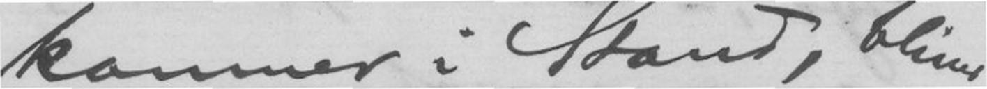kommer i Stand, bliver
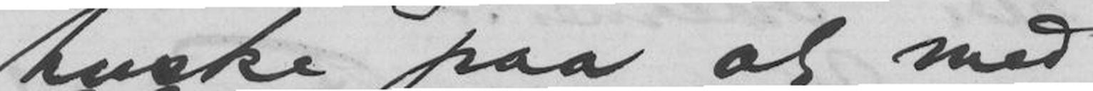huske paa at med
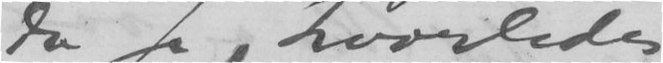da se, hvorledes
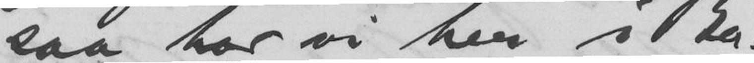saa har vi her i Ber-
